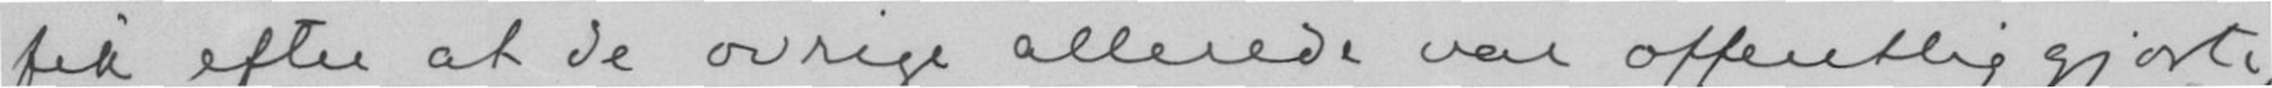fik efter at de øvrige allerede var offentliggjort,
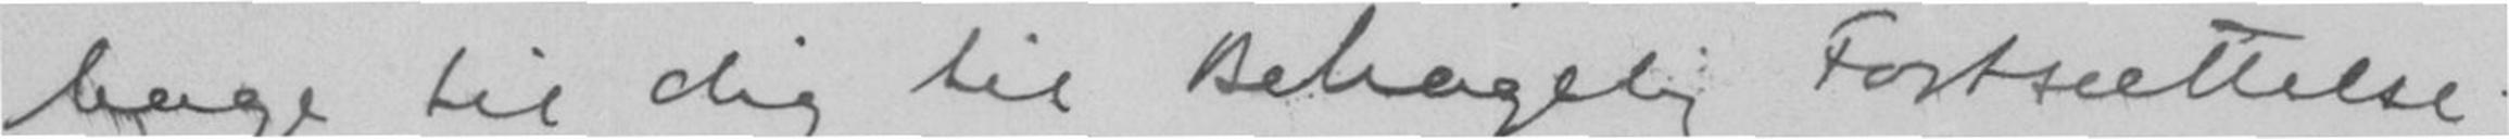bage til dig til Behagelig Fortsættelse.
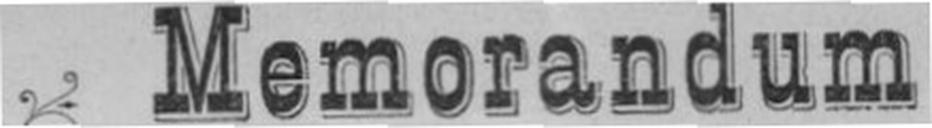Menorandum
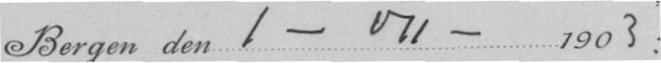Bergen den1 - VII - 1903
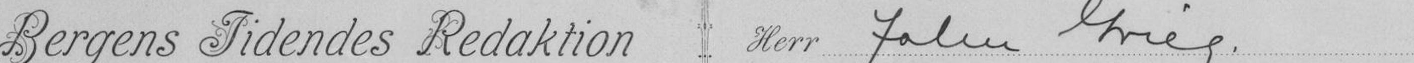Bergens Tidendes Redation Herr John Grieg.
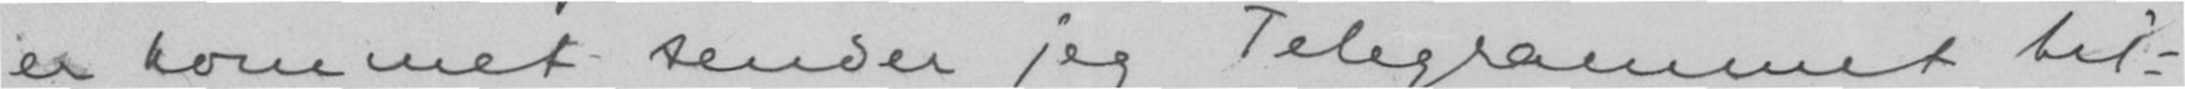er kommet sender jeg Telegrammet til-
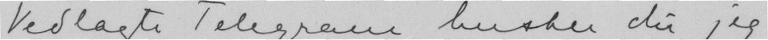Vedlagte Telegram husker du jeg
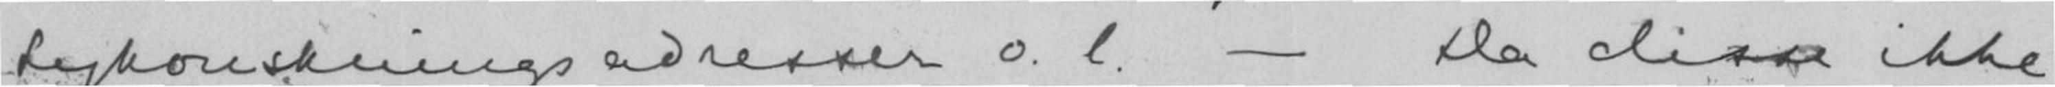Lykønskningsadresser o. l. - Da disse ikke
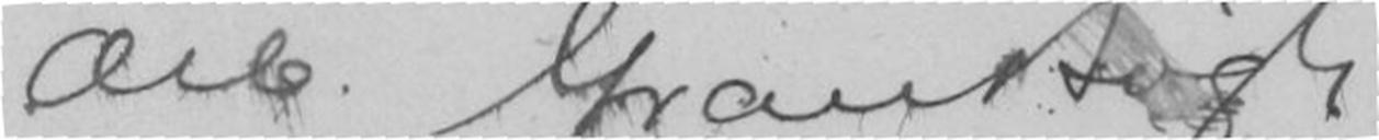Ærb. Gran Bøgh
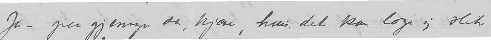Ja – paa gjensyn da, kjære, hvis det kan lage sig slik
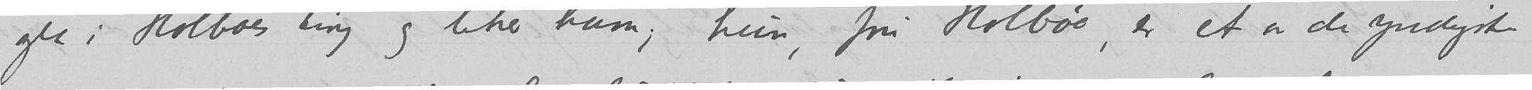gla i Holbøes ting og liker ham; hun, fru Holbøe, er et av de yndigste
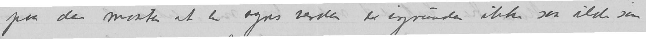paa den maaten at en syns verden er igrunden ikke saa ilde som
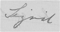Sigrid.
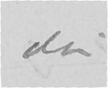din
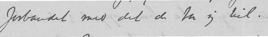forbandet med det de tar sig til.
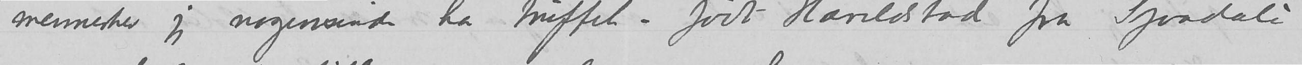mennesker jeg nogensinde har truffet – født Harildstad fra Sjaadali
